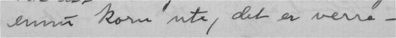ennu korn ute, det er verre —
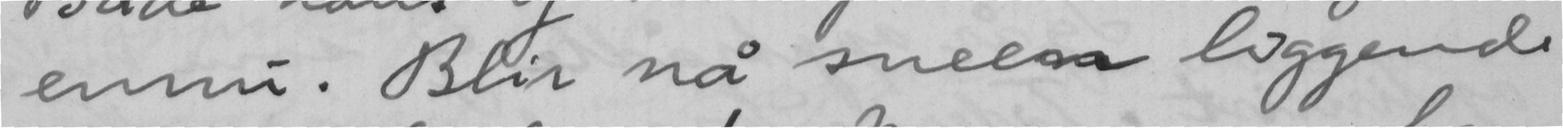ennu. Blir nå sneen liggende
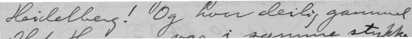Heidelberg! Og hvor deilig gammel
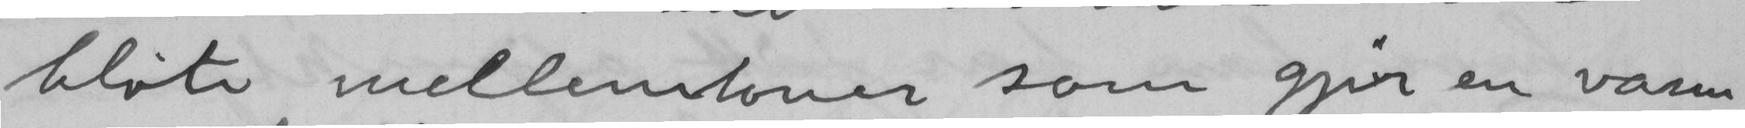bløte mellemtoner som gjør en varm
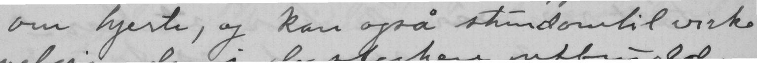om hjerte, og kan også stundomtil virke
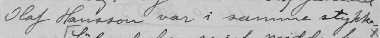Olaf Hansson var i samme stykke.
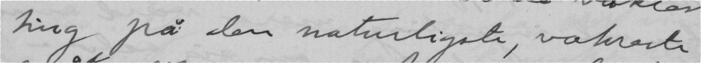ting på den naturligste, vakreste
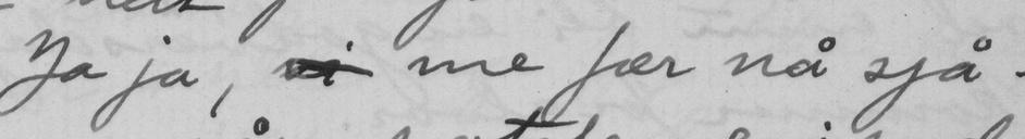Ja ja, vi me fær nå sjå.
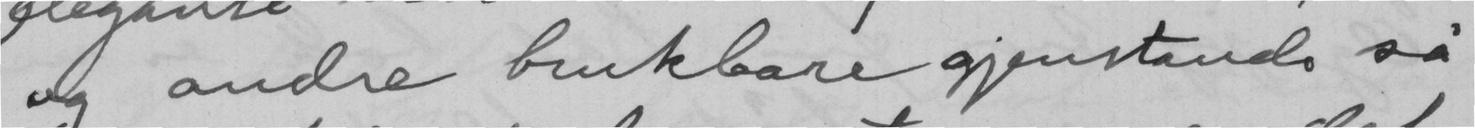og andre brukbare gjenstands så
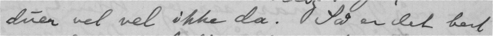duer vel vel ikke da.  Så er det best
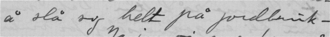å slå sig helt på jordbruk -
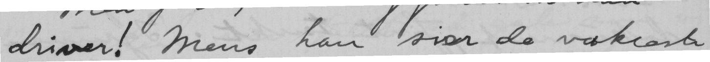driver! Mens han sier de vakreste
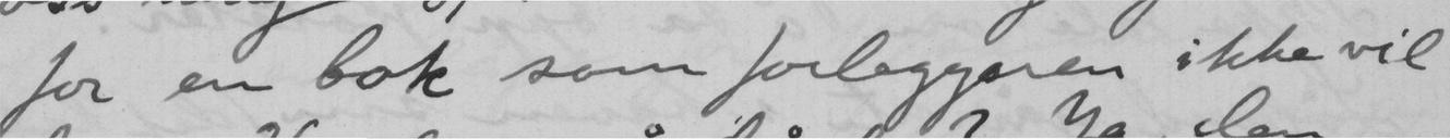for en bok som forleggeren ikke vil
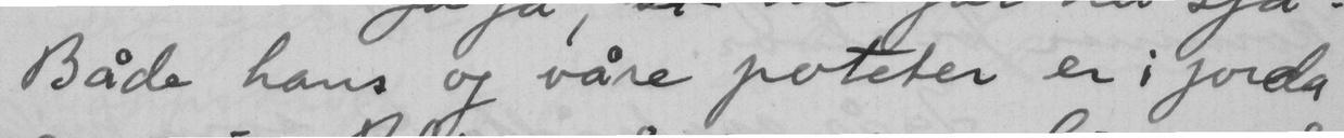Både hans og våre poteter er i jorda
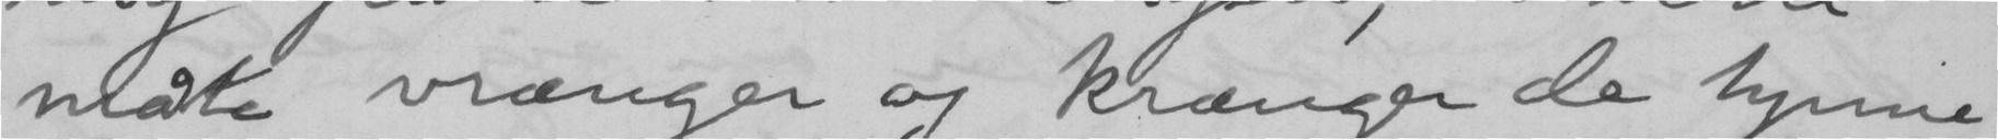måte vrænger og krænger de tynne
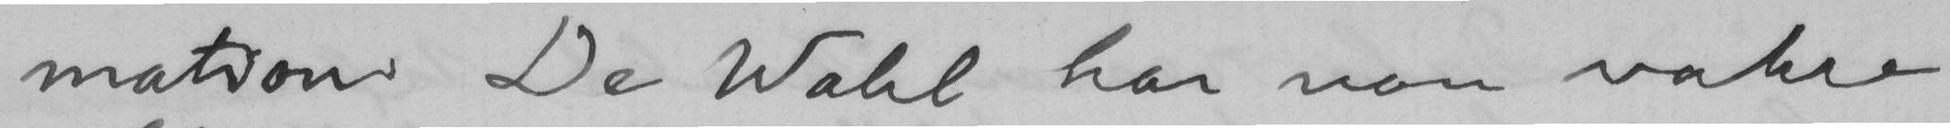mationer. De Wahl har noen vakre
